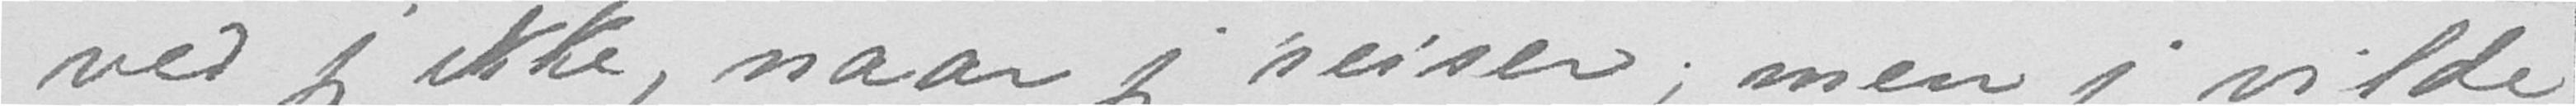ved jeg ikke, naar jeg reiser; men jeg vilde
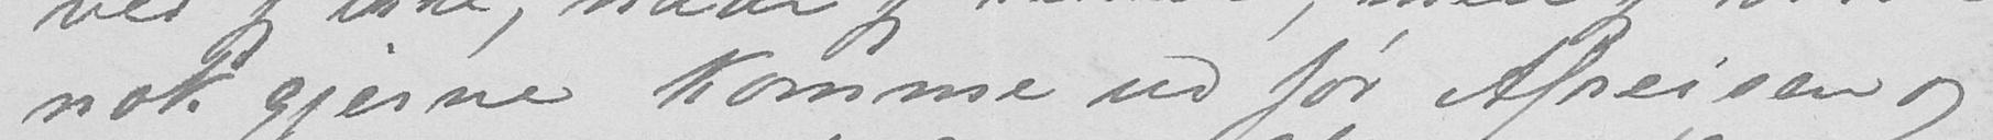nok gjerne komme ud før Afreisen og
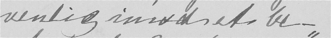venlig imod et be-
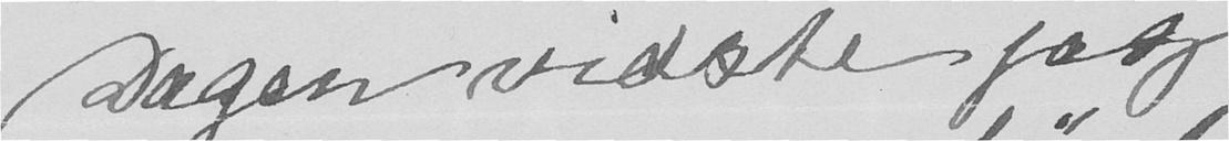Dagen vidste jeg
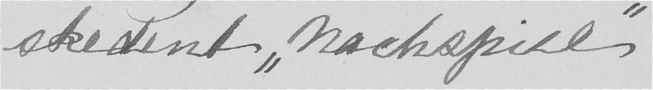skedent "Nachspiel"
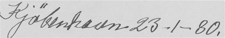Kjøbenhavn 23-1-80.
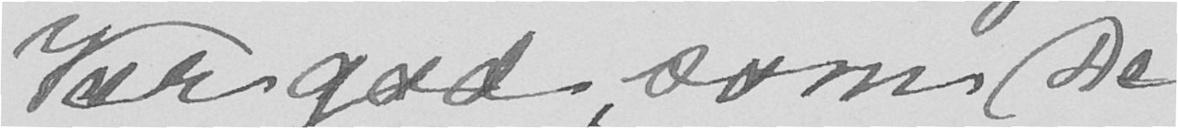Vær god, som De
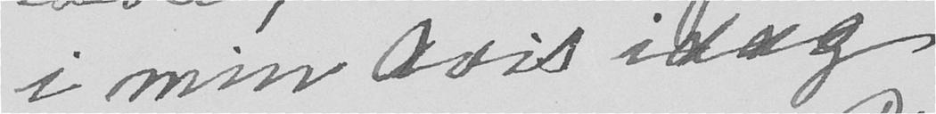i min Avis idag.
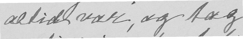altid var, og tag
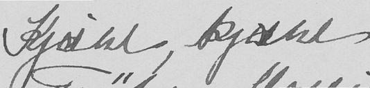Kjære, kjære
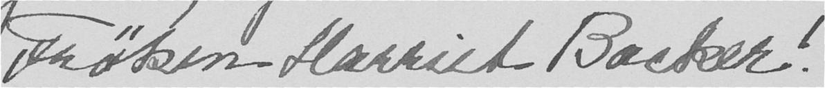Frøken Harriet Backer!
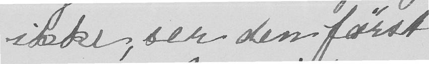ikke, ser den først
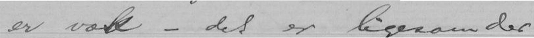er væk - det er ligesom der
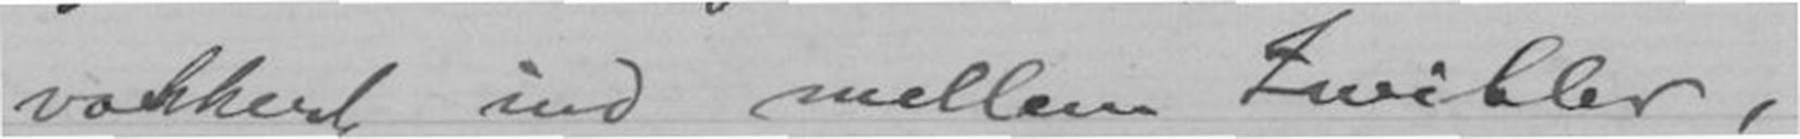vakkert ind mellem Zwibler,
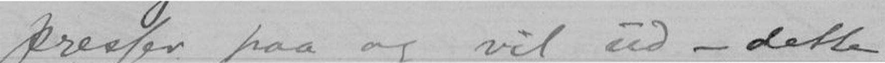præsser paa og vil ud - dette
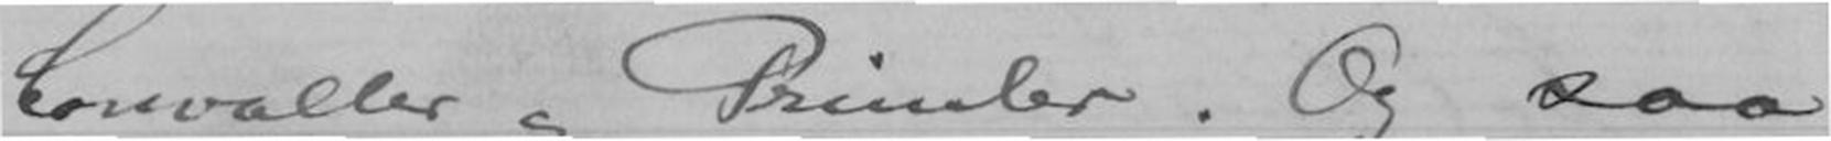Convaller og Primler. Og saa
